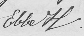Ebbe H.
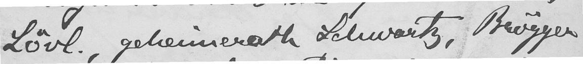Løvl., geheimerath Schwartz, Brøgger
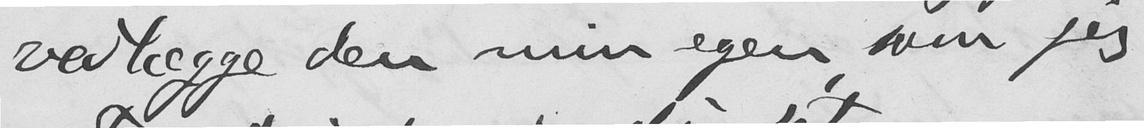vedlægge den min egen, som jeg
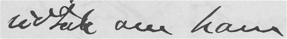udtale om ham.
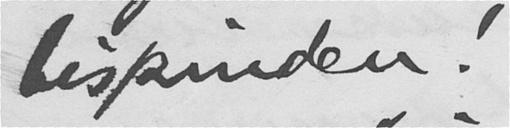bispinden!
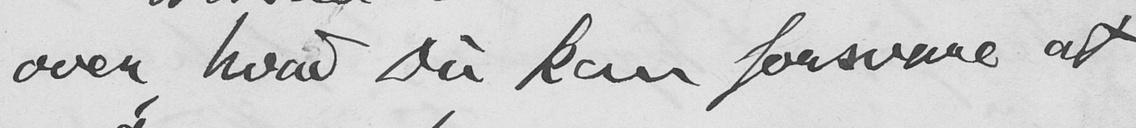over, hvad Du kan forsvare at
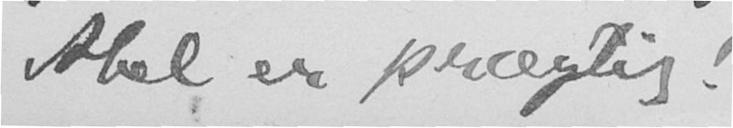Abel er prægtig!
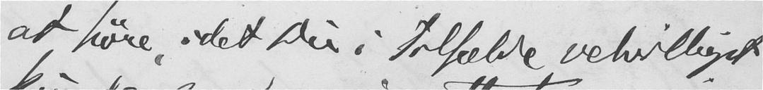at høre, idet Du i tilfælde velvilligst
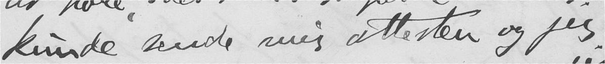kunde sende mig attesten og jeg
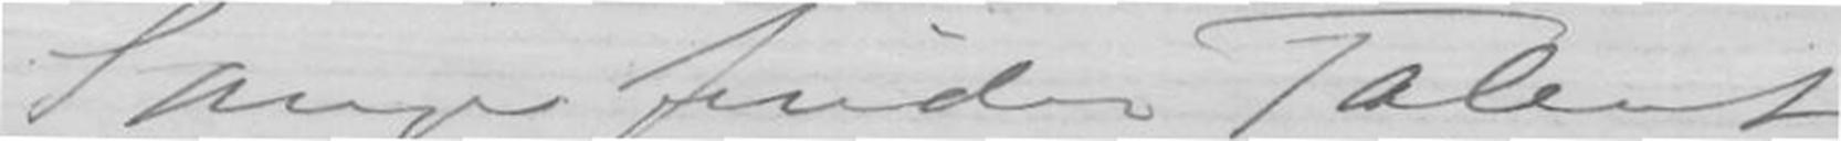Sange finder Talent
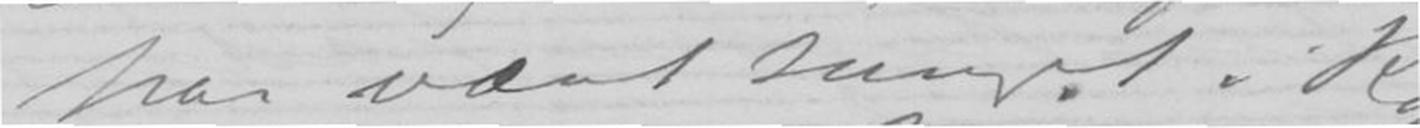har været sunget i Rom
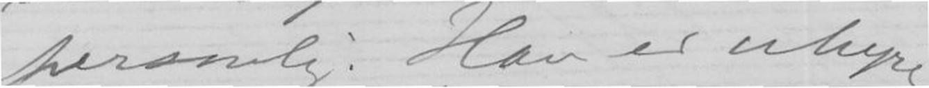personlig: Han er uhyre
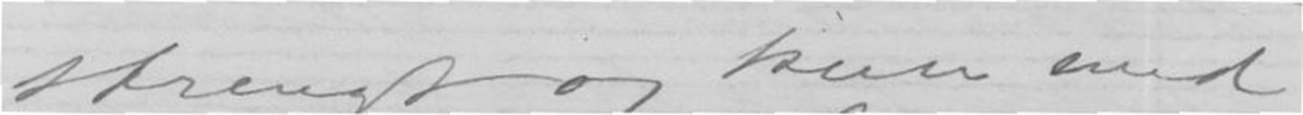strengt og kun med
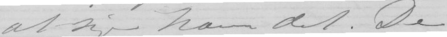at sige ham det. De
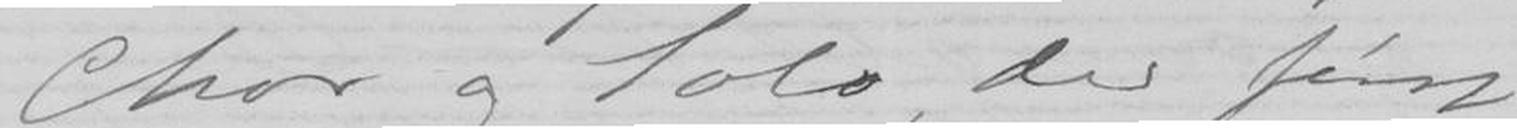Chor og Solo, der først
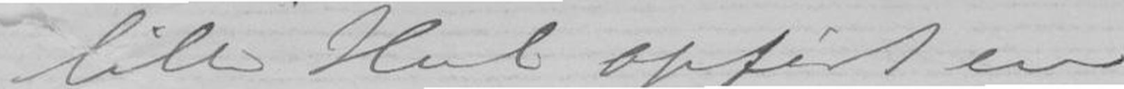lille Hul opført en
